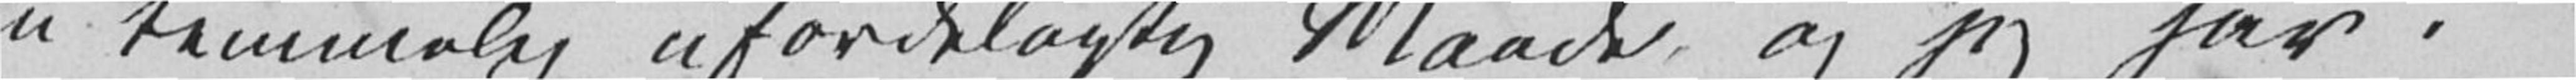en temmelig ufordelagtig Maade, og jeg har i
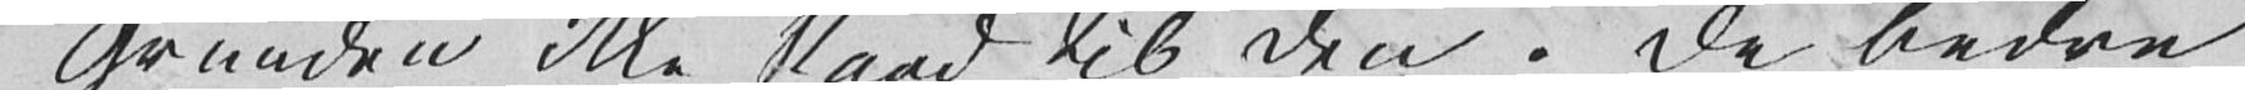Grunden ikke Raad til den. De bedre
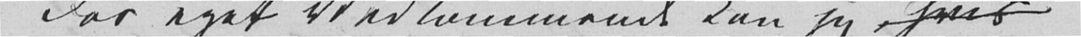For eget Vedkommende kan jeg, hvis
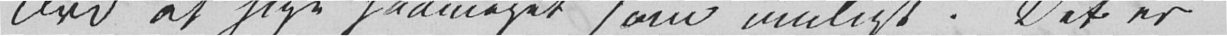Ord at sige saameget som muligt. Det er
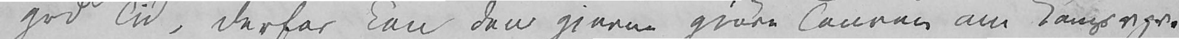god Tid, derfor kan den gjerne gjøre Touren om Kongsvgr.
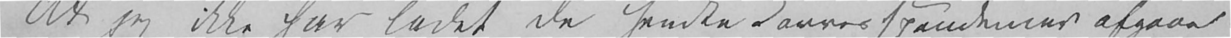At jeg ikke har ladet de sendte Korresspondencer afgaae
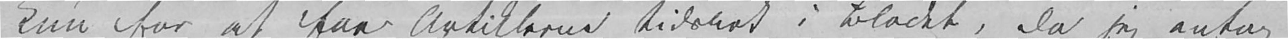kun for at faa Artiklerne tidsnok i Bladet, da jeg antog
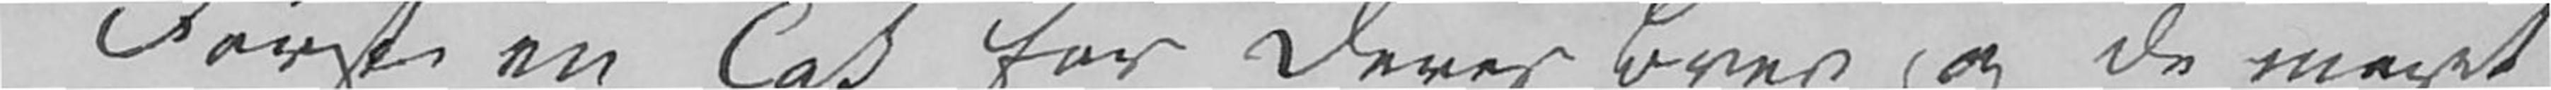Først en Tak for Deres Brev og de meget
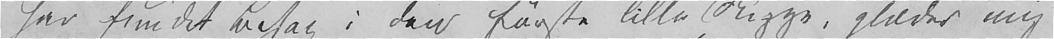har fundet Behag i den første lille Skizze, glæder mig
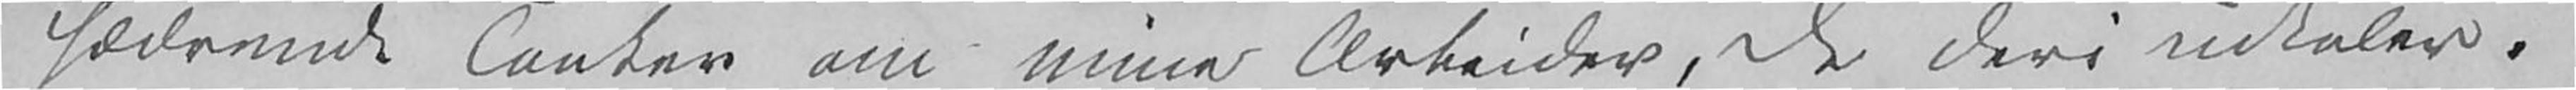hædrende Tanker om mine Arbeider, De deri udtaler.
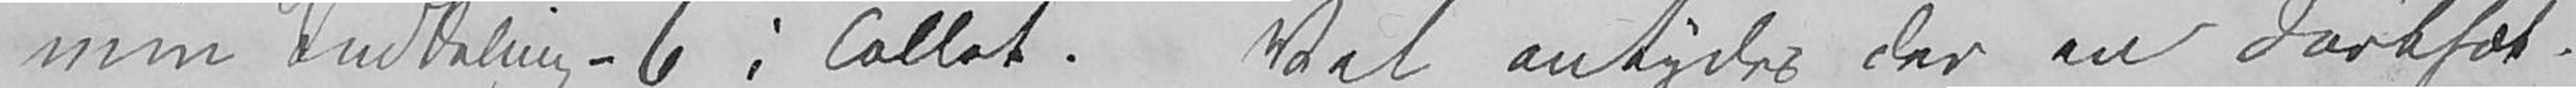min Inddeling – 6 i Tallet. Vel antydes der en Fortsæt¬
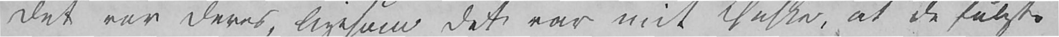det var Deres, ligesom det var mit Ønske, at de fulgte
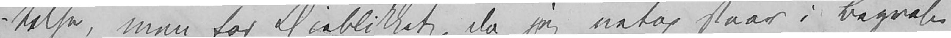telse, men for Øieblikket, da jeg netop staar i Begreb
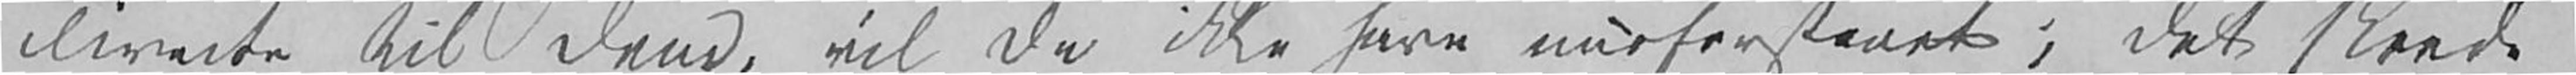directe til Dem, vil De ikke have misforstaaet; det skeede
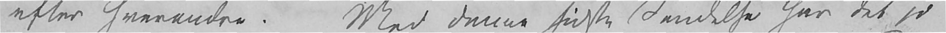efter hverandre. Med denne sidste Sendelse har det jo
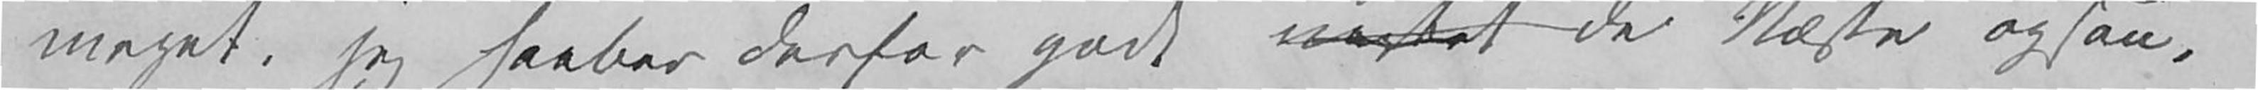meget, jeg haaber derfor godt uagtet de Næste ogsaa,
